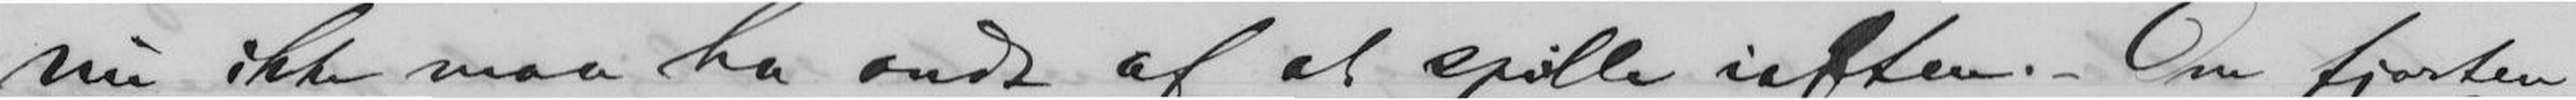nu ikke maa ha ondt af at spille iaften. - Om fjorten
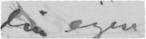Din egen
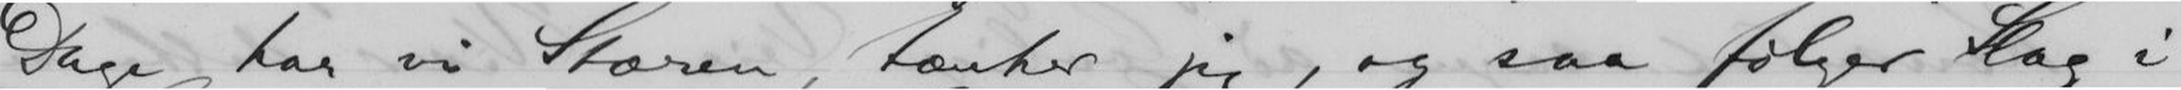Dage har vi Stæren, tænker jeg, og saa følger Slag i
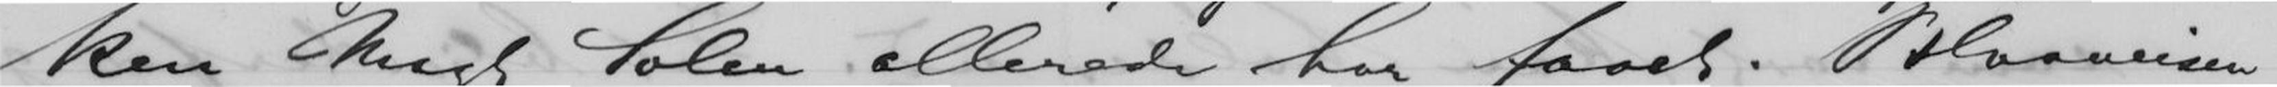ken Magt Solen allerede har faaet. Blaaveisen
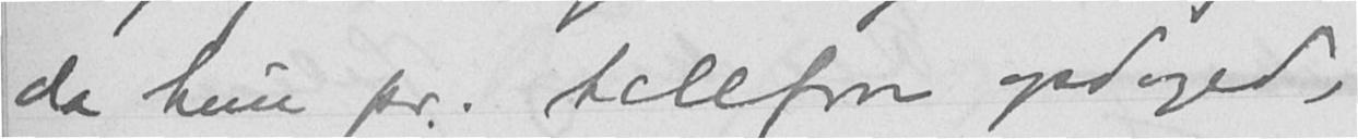da hun pr. telefon opdaged,
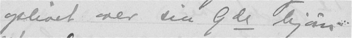oplivet over sin 9de bjørn.
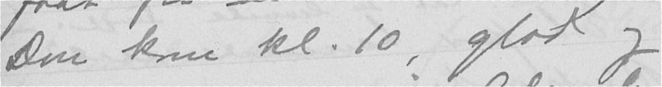Don kom kl. 10, glad og
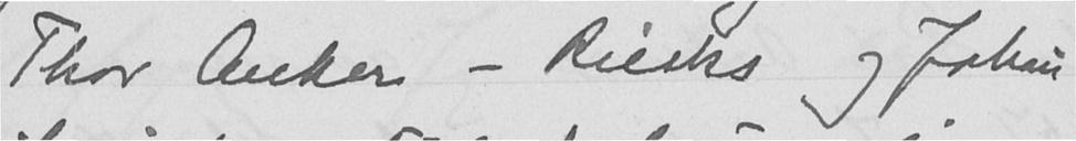Thor Anker - Riecks og Johan
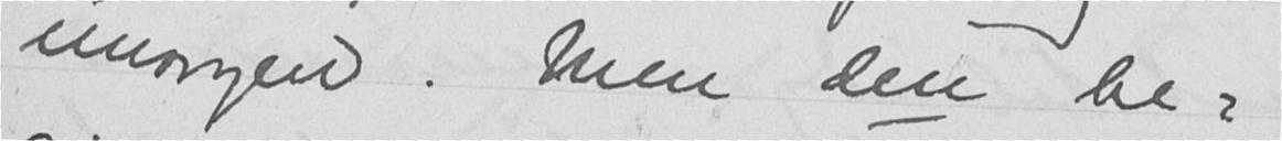imorgen. Men den be-
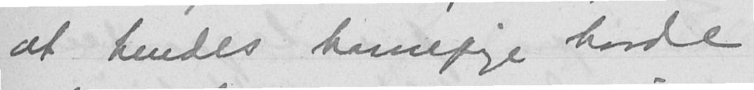at hendes barnepige havde
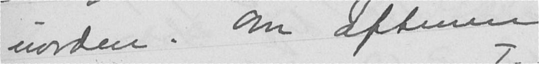uorden. Om aftenen
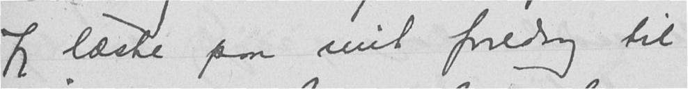Jeg læste paa mit foredrag til
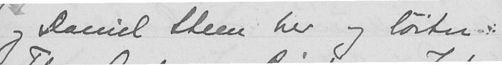og Daniel Steen her og løitn.
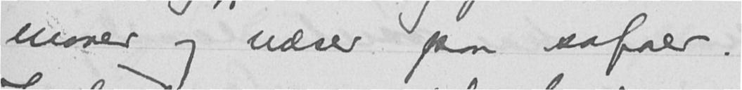maver og næser paa sofaer.
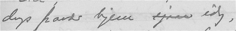dags fravær hjem igaar idag,
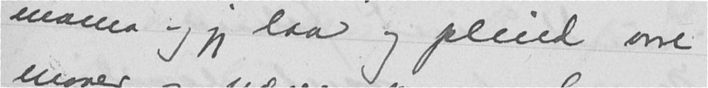mama og jeg laa og pleied vore
